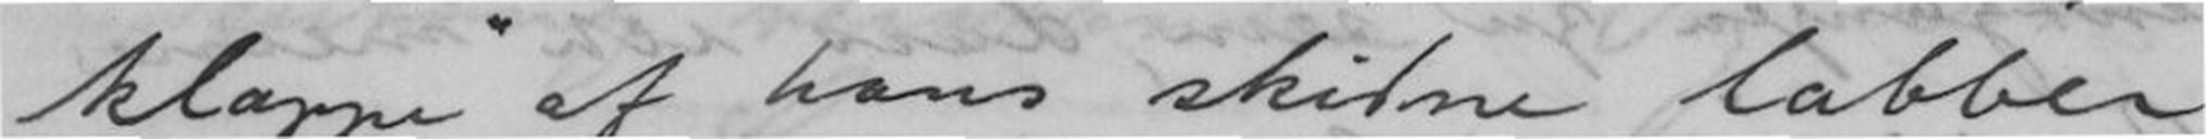"klappe" af hans skidne labber
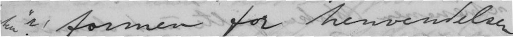formen for henvendelsen
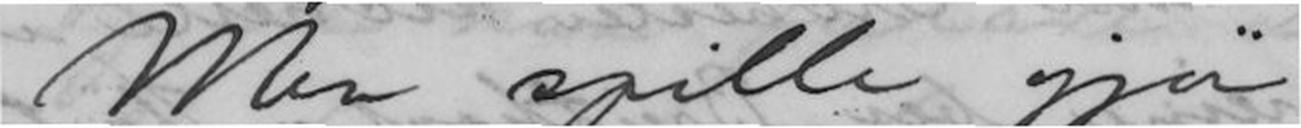Men spille gjör jeg,
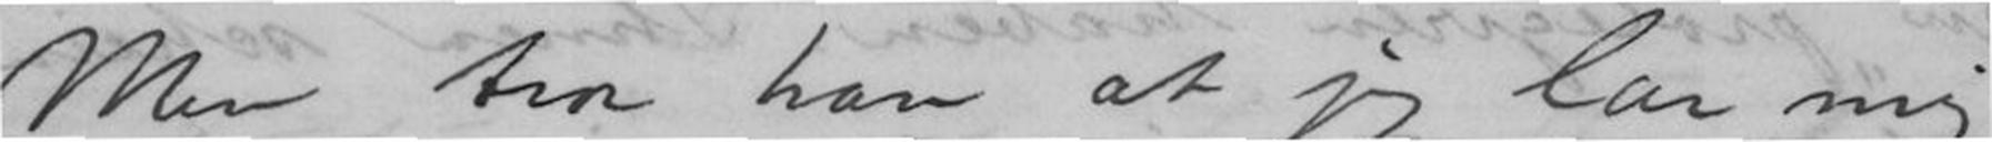Men tror han at jeg lar mig
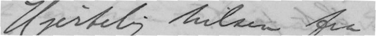Hjertelig hilsen fra
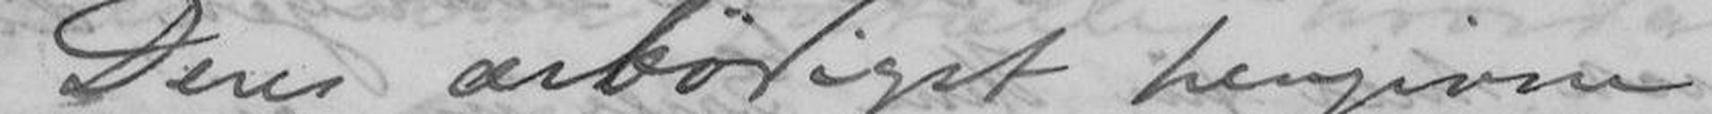Deres ærbödigst hengivne
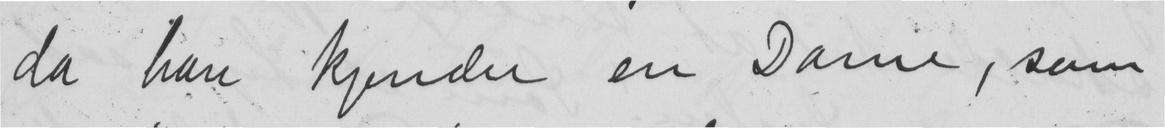da han kjender en Dame, som
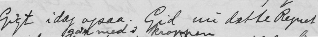Gigt idag ogsaa. Gid nu dette Regnet
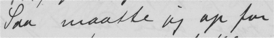Saa maatte jeg op for
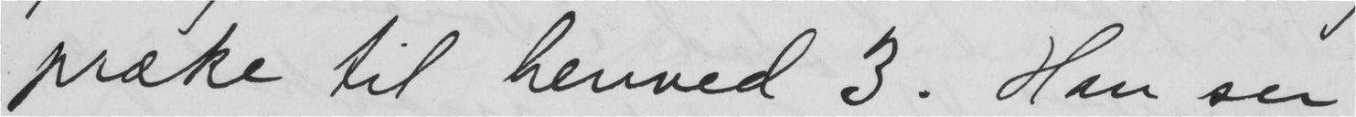præke til henved 3. Han ser
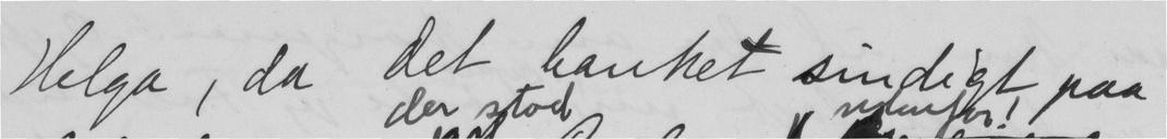Helga, da det banket sindigt paa
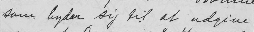som byder sig til at udgive
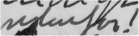nedenfor!
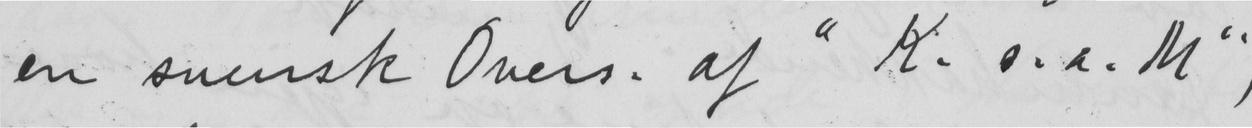en svensk Overs. af " K. s. a. M.",
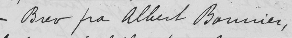- Brev fra Albert Bonnier,
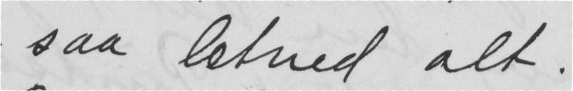saa letned alt.
